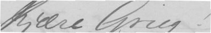Kjære Grieg!
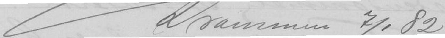Drammen 7/1 82
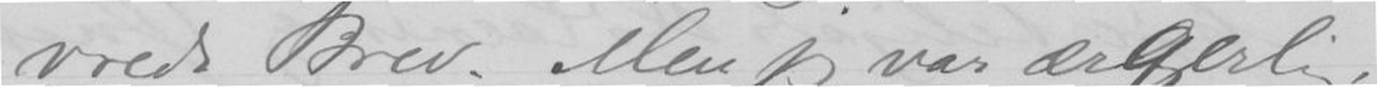vreds Brev. Men jeg var ærgerlig,
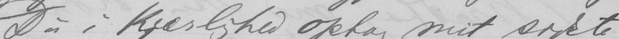Du i kjærlighed optog mit sidste
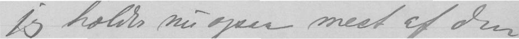jeg holder nu ogsaa mest af den
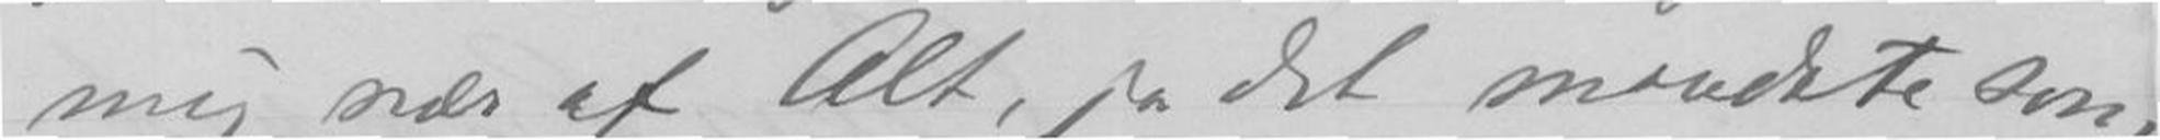mig nær af Alt, ja det mindste som
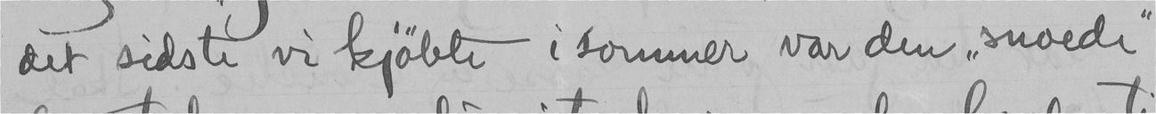det sidste vi kjøbte i sommer var den "snoede"
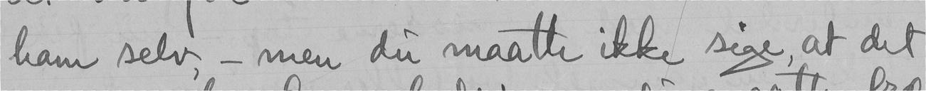ham selv, - men du maatte ikke sige, at det
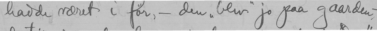hadde været i før, - den "blev" jo paa gaarden,-
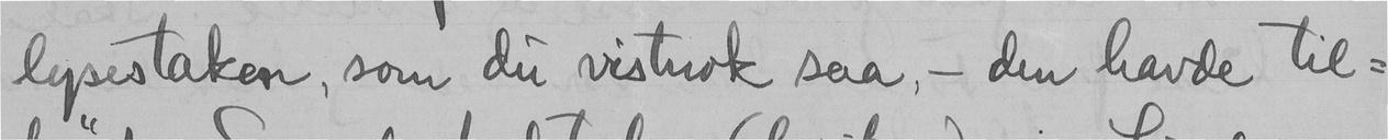lysestaken, som du vistnok saa, - den havde til-
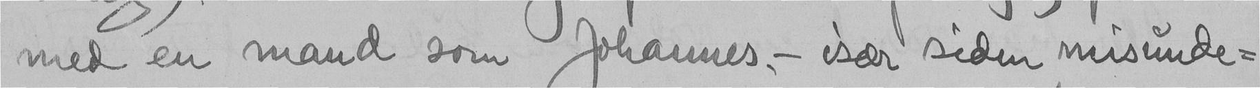med en mand som Johannes, - især siden misunde-
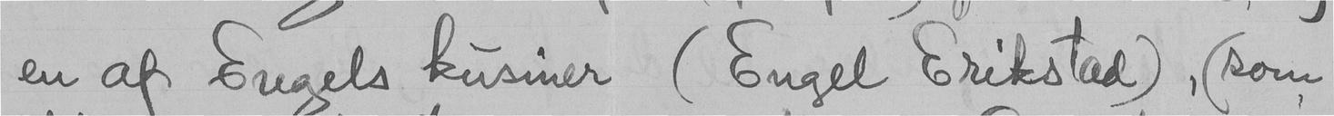en af Engels kusiner (Engel Erikstad), (som
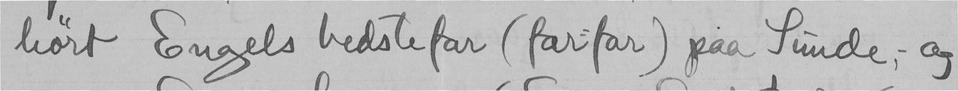hørt Engels bedstefar (farfar) paa Sunde, - og
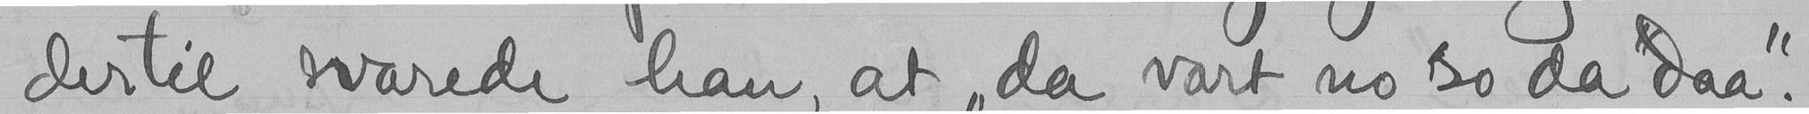dertil svarede han, at "da vart no so da daa".
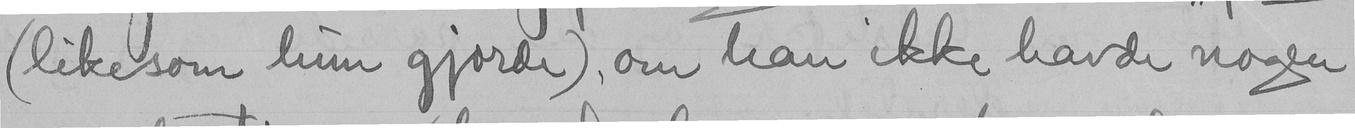(likesom hun gjorde), om han ikke havde nogen
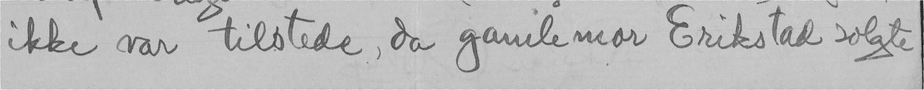ikke var tilstede, da gamle mor Erikstad solgte
